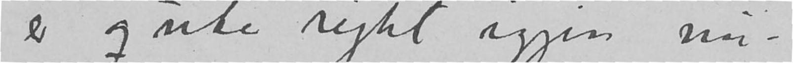er quite right igjen nu –
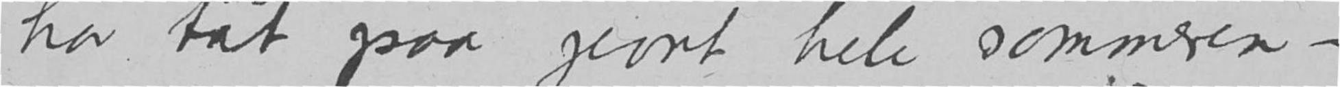har tat paa jevnt hele sommeren –
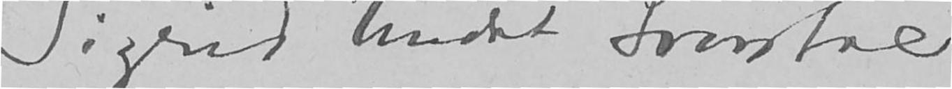Sigrid Undset Svarstad
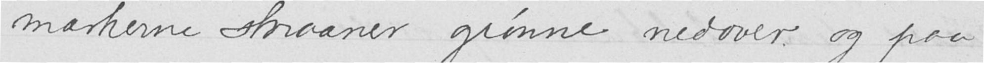markerne skraaner grønne nedover og paa
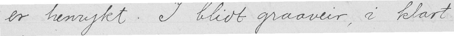er henrykt. I blidt graaveir, i klart
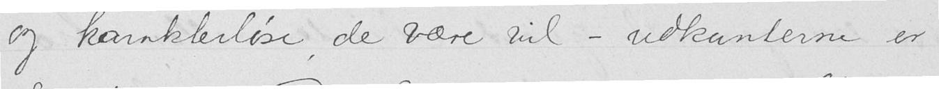og karakterløse, de være vil — udkanterne er
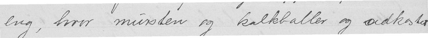eng, hvor mursten og kalkballer og udkastet
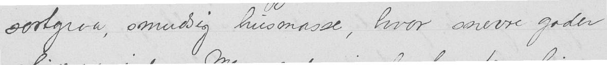sortgraa, smudsig husmasse, hvor snevre gader
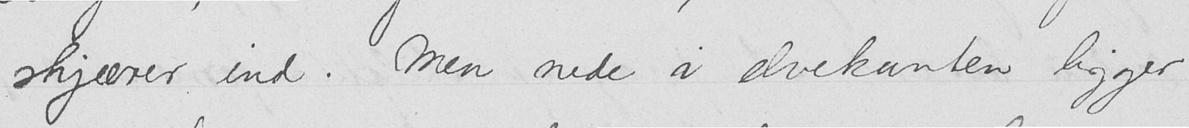skjærer ind. Men nede i elvekanten ligger
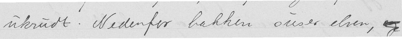ukrudt. Nedenfor bakken suser elven, og
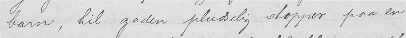barn, til gaden pludselig stopper paa en
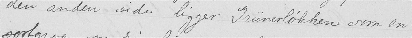den anden side ligger Grünerløkken som en
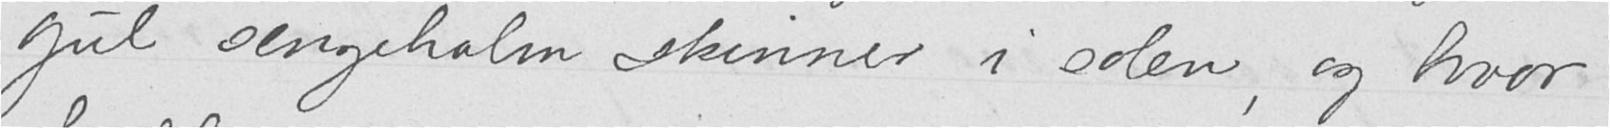gul sengehalm skinner i solen, og hvor
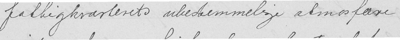fattigkvarterets ubestemmelige atmosfære
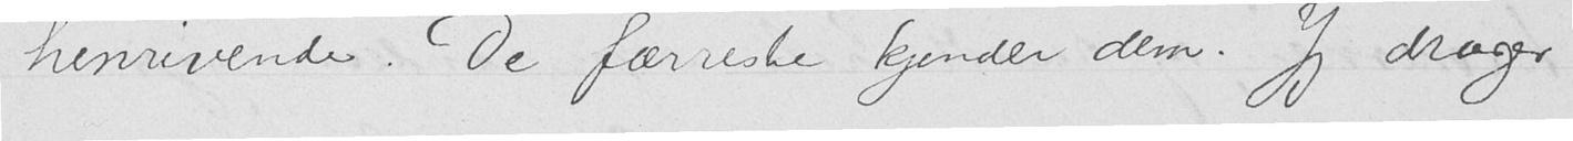henrivende. De færreste kjender dem. Jeg drager
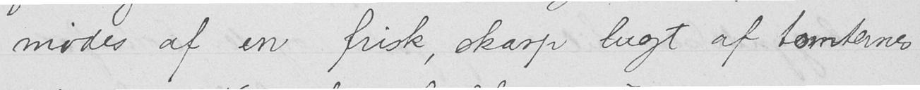mødes af en frisk, skarp lugt af tomternes
